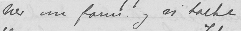her om form. og vi talte
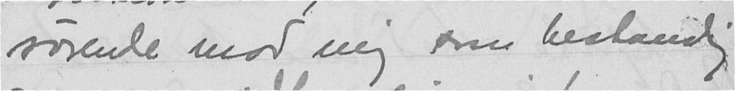rørende mod mig som bestandig.
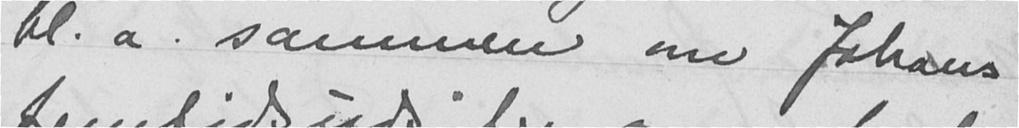bl. a. sammen om Johans
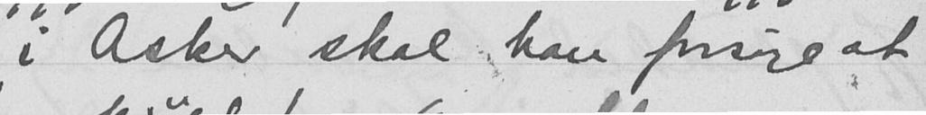i Asker skal han forsøge at
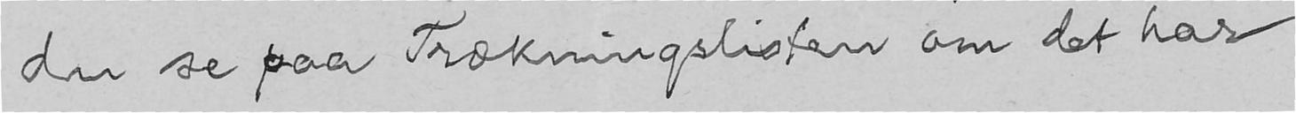du se paa Trækningslisten om det har
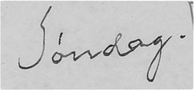Søndag.
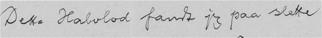Dette Halvlod fandt jeg paa slette
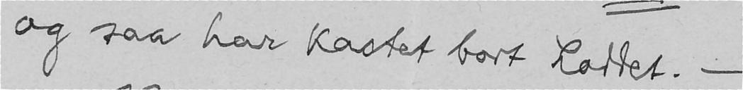og saa har kastet bort Loddet. -
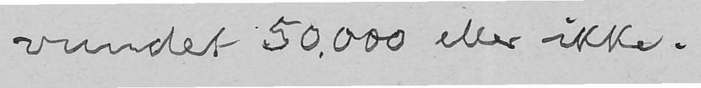vundet 50.000 eller ikke.
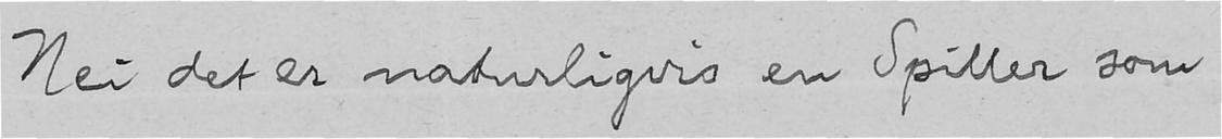Nei det er naturligvis en Spiller som
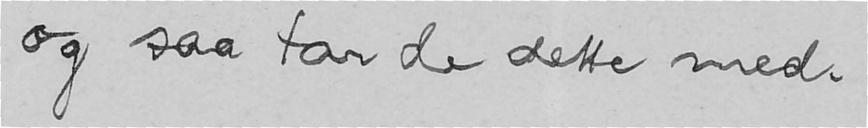og saa tar de dette med.
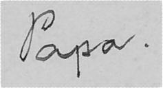Papa.
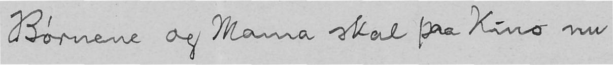Børnene og Mama skal paa Kino nu
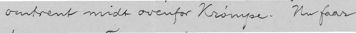omtrent midt ovenfor Krømpe. Nu faar
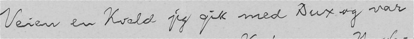Veien en Kveld jeg gik med Dux og var
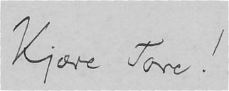Kjære Tore!
